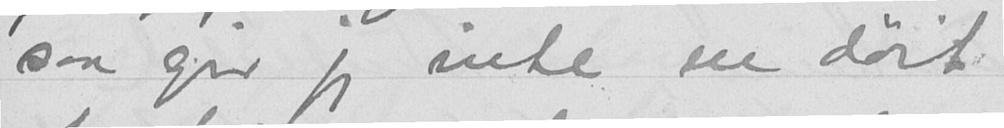saa gør jeg inte en døit
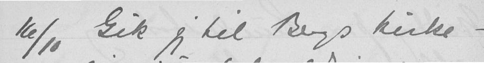16/10 Gik jeg til Bergs kirke -
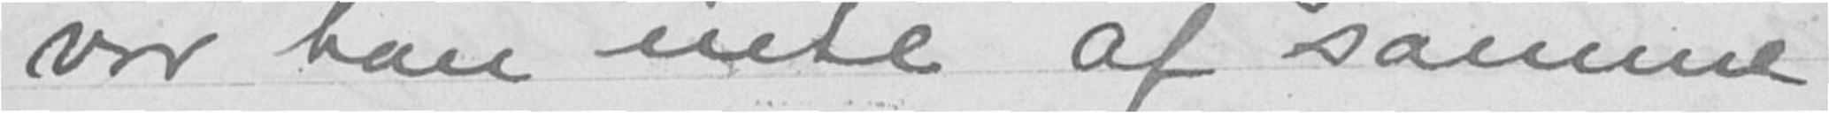var han inte af samme
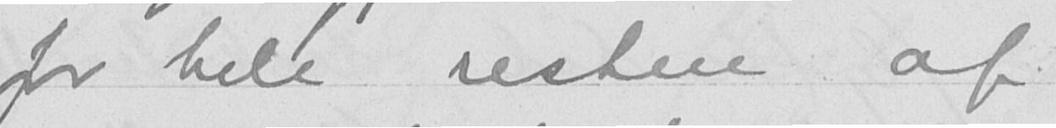for hele resten af
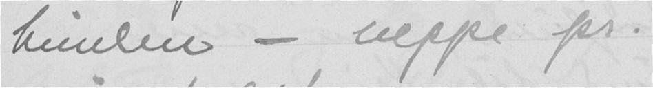himlen - neppe pr.
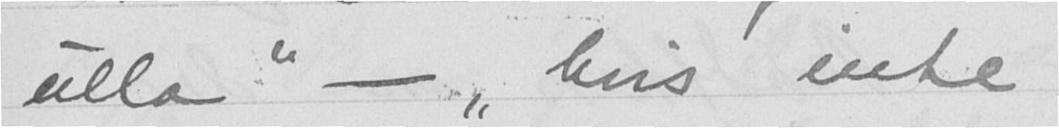ulla" - "hvis inte
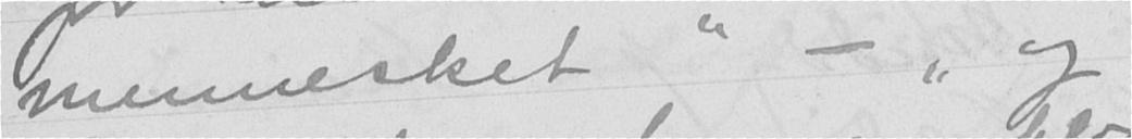mennesket" - "og
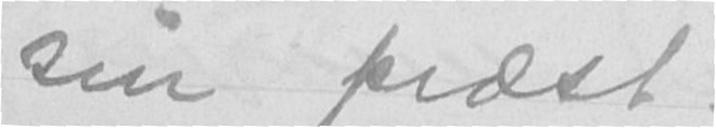sin præst.
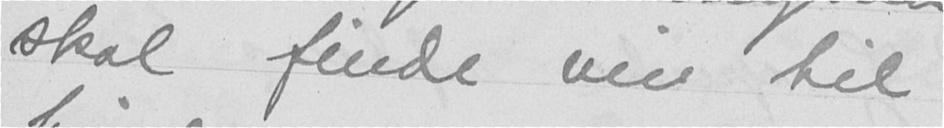skal finde vei til
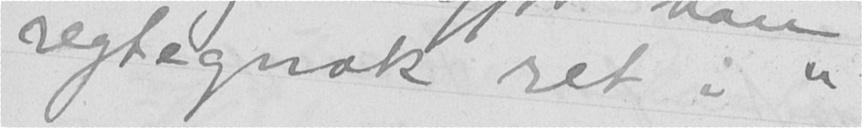regtegnok ret i"
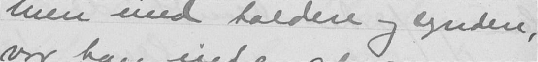men med toldere og syndere,
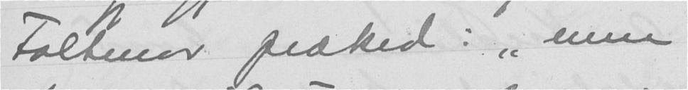Foltmar præked: "men
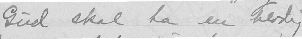Gud skal ta en blodig
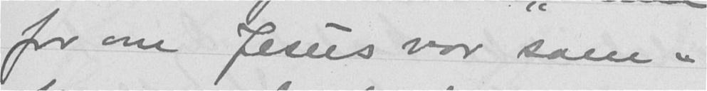for om Jesus var sam-
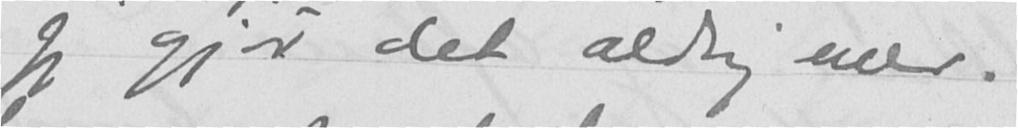jeg gjør det aldrig mer.
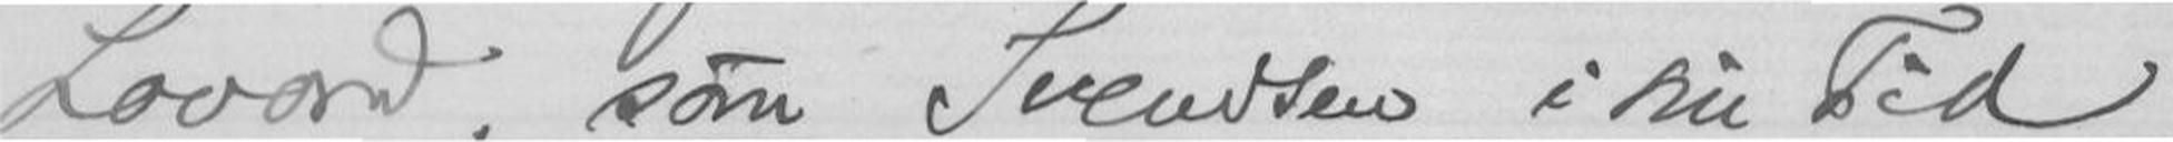Lovord, som Svendsen i nu Tid
